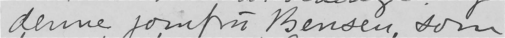denne jomfru Bensen, som
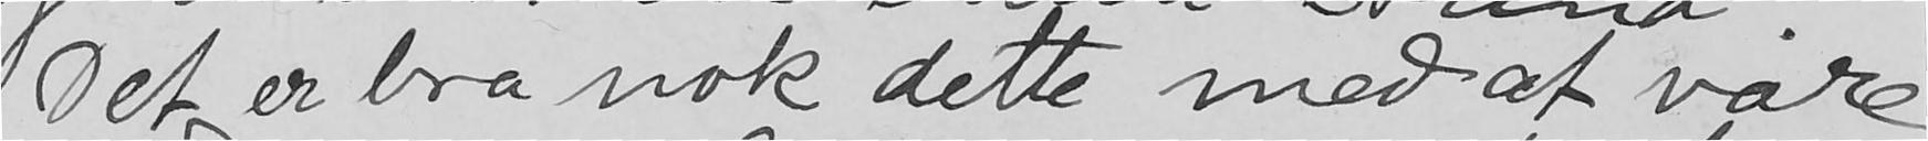Det er bra nok dette med af være
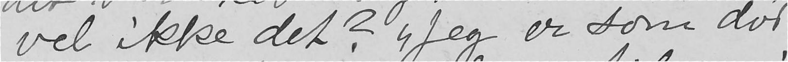vel ikke det? "Jeg er som død
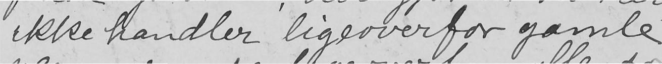ikke handler ligeoverfor gamle
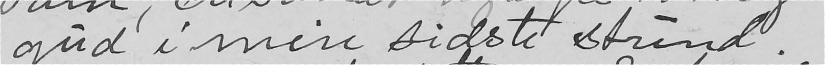gud i min sidste stund.
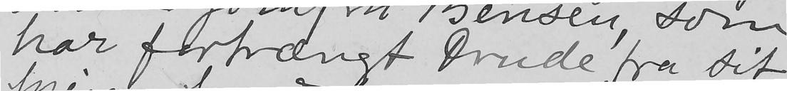har fortrængt Drude fra sit
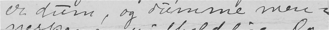er dum, og dumme men-
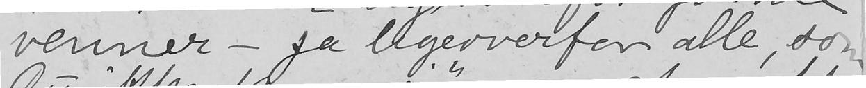venner - ja ligeoverfor alle, som
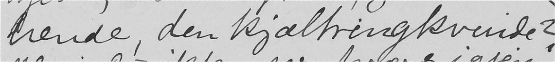hende, den kjæltringkvinde?
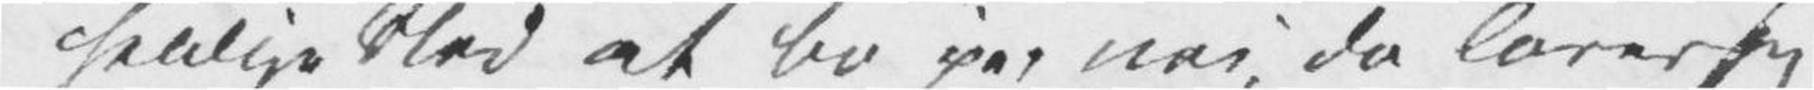heldige Sted at bo på, nei, da lover jeg
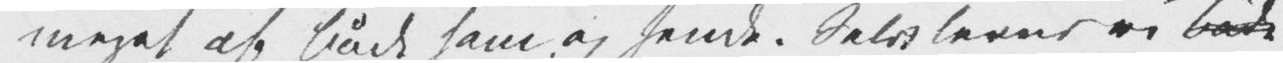meget af både ham og hende. Selv lever vi både
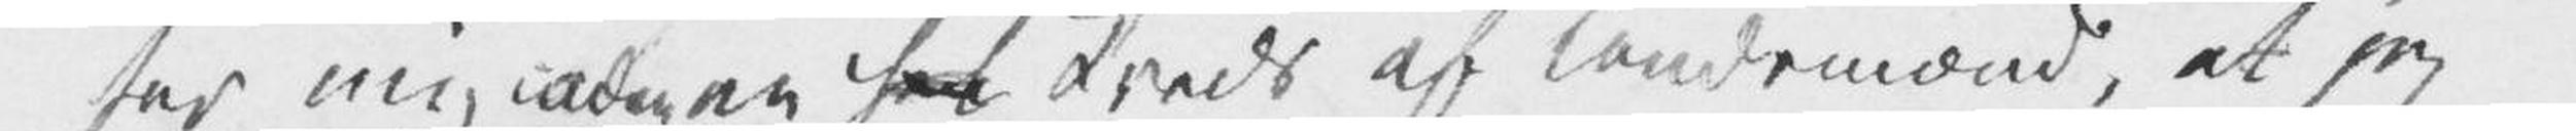for mig uden en hel Kreds af Landsmænd, at jeg
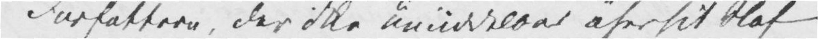Forfattere, der ikke umiddelbart øser sit Stof
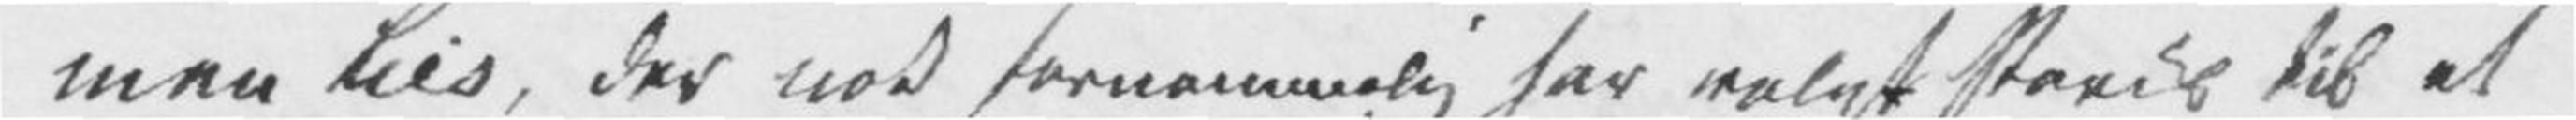men Lies, der nok fornemmelig har valgt Paris til et
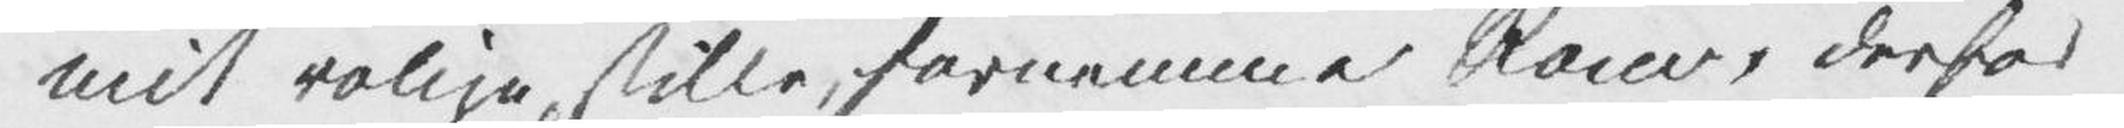mit rolige, stille, fornemme Rom, derfor
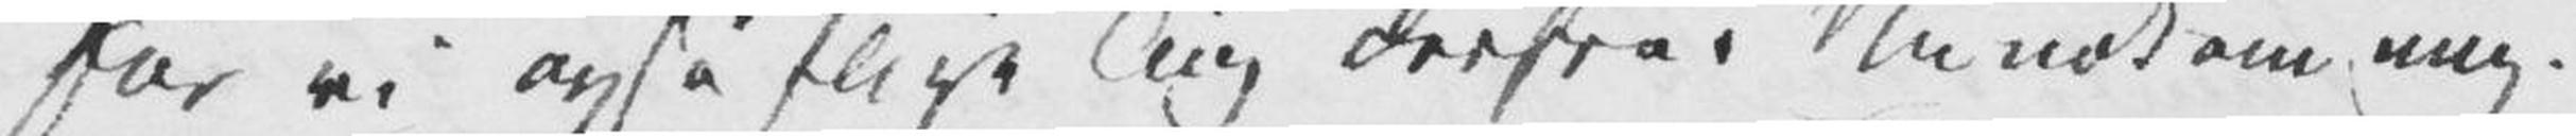får vi også slige Ting derfra. Nu nok om mig.
